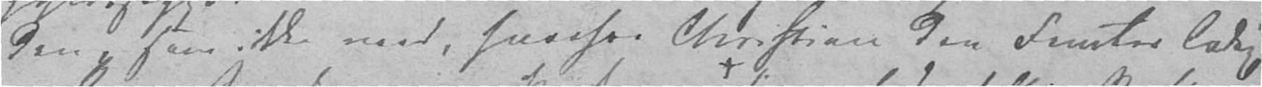den, som ikke veed, hvorfor Christian den Femtes Codex
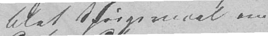blot Spørgsmaal om
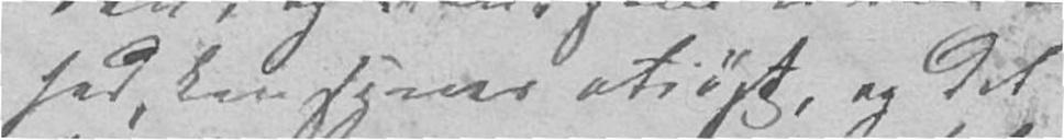hed, kan synes otiøst, og det
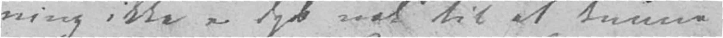ning ikke er dyb nok til at kunne
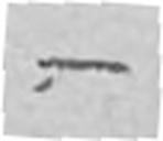.–
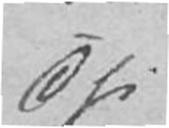Thi
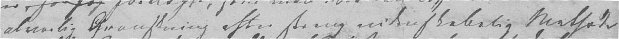alvorlig Granskning efter streng videnskabelig Methode
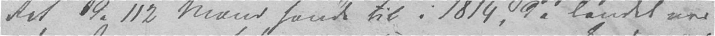Ret de 112 Mand havde til i 1814, da Landet var
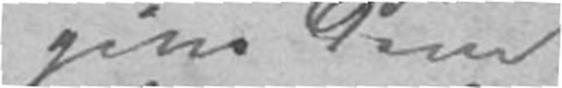give dem
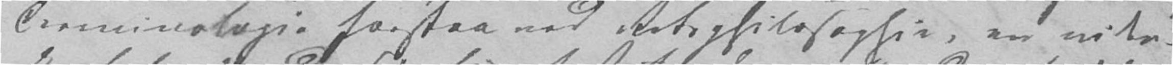Terminologie forstaa ved Retsphilosophie, en viden-
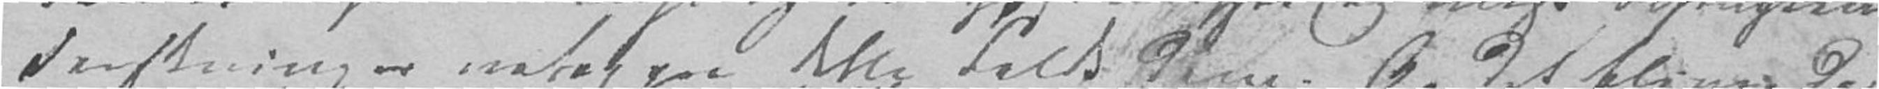Forskninger netop paa dette Feldt dem. Og det blive da
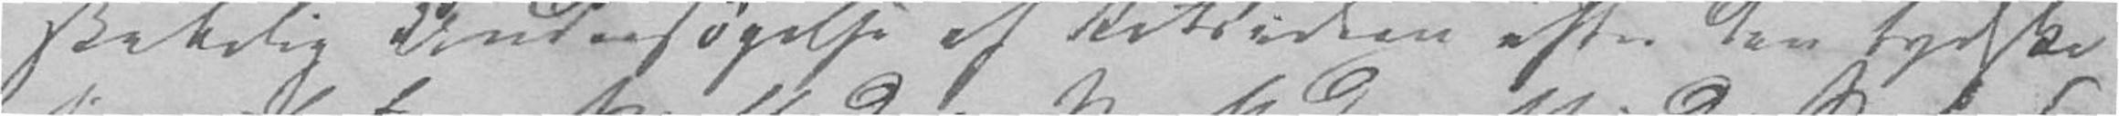skabelig Undersøgelse af Retsideen efter den tydske
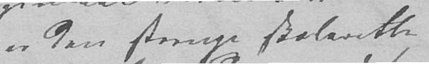er den strænge skolerette
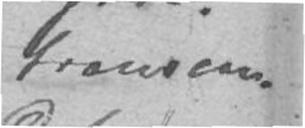transcen-
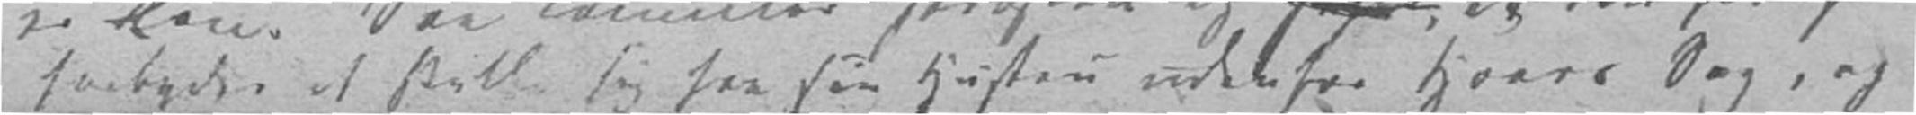forbyder at skille sig fra sin Hustru udenfor Hoers Sag, og
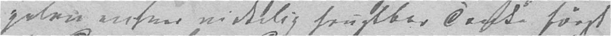gelen enhver virkelig frugtbar Tanke først
